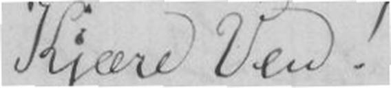Kjære Ven!
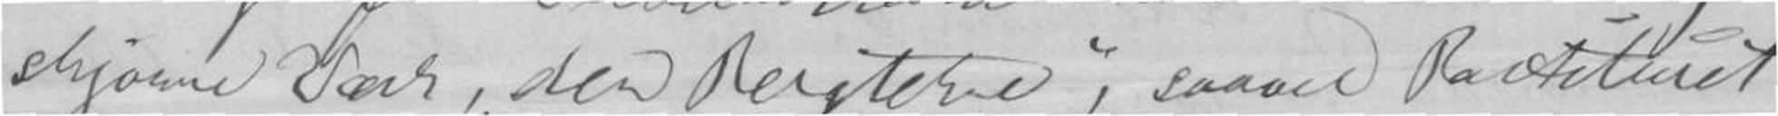skjønne Værk, den Bergtekne, saavel Partituret
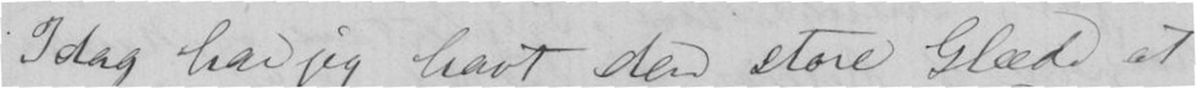Idag har jeg havt den store Glæde at
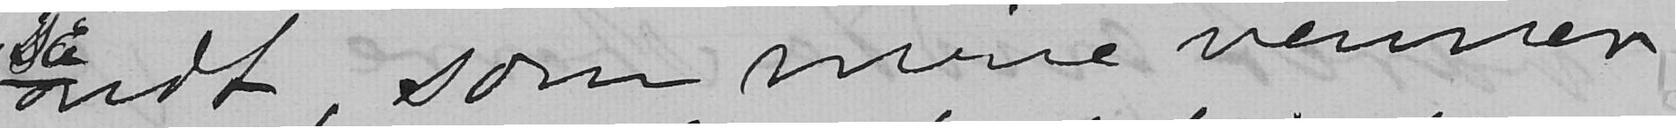ondt, som mine venner
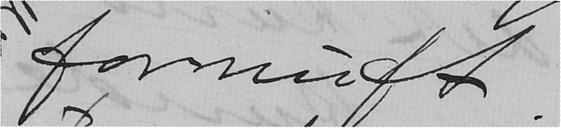fornuft.
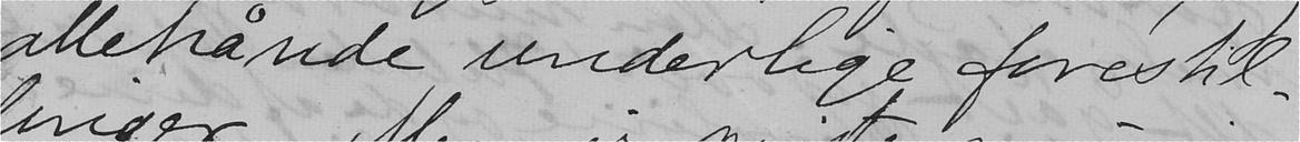allehånde underlige forestil-
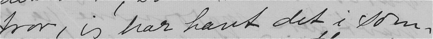tror, jeg har havt det i som-
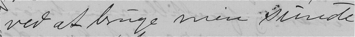ved at bruge min sunde
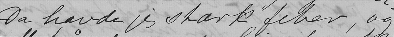Da havde jeg stærk feber, og
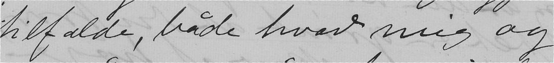tilfælde, både hvad mig og
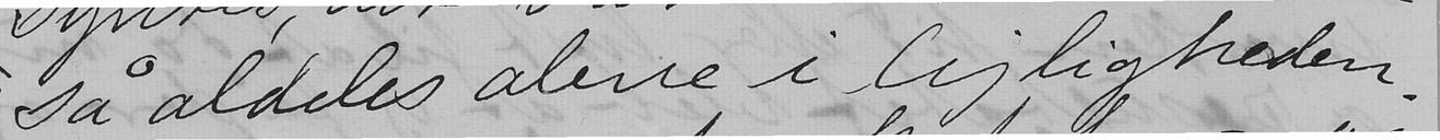så aldeles alene i lejligheden.
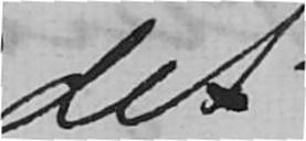det
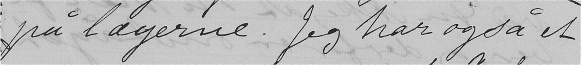på lægerne. Jeg har også et
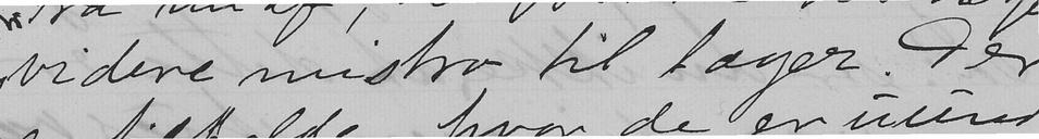videre mistro til læger. Der
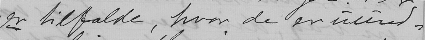er tilfælde, hvor de er uund-
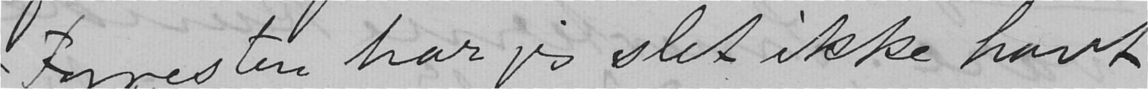Forresten har jeg slet ikke havt
